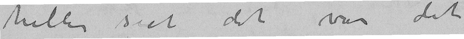heller seet det var det
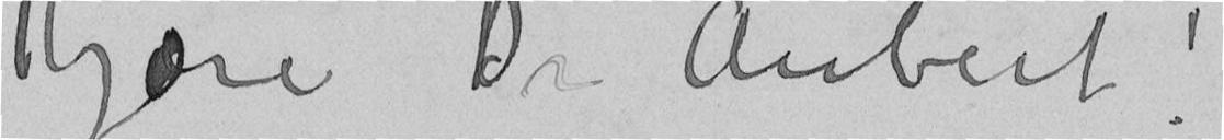Kjære Dr Aubert!
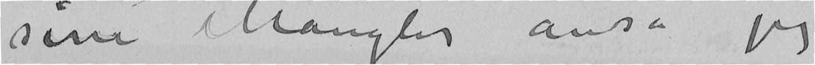sine Mangler anså jeg
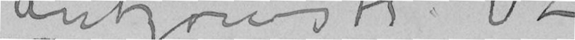Lützowstr 82
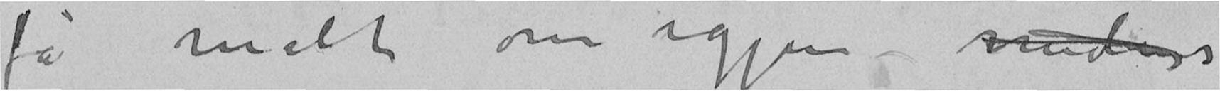få malt om igjen – medens
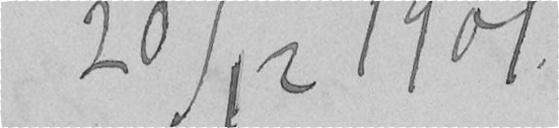20/12 1901
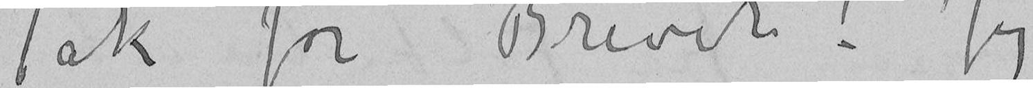Tak for Brevet! Jeg
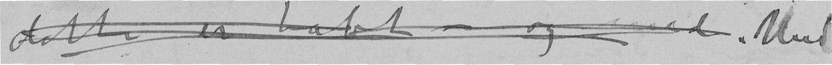dette er tabt – og Med
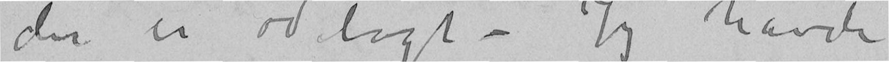der er ødelagt – Jeg havde
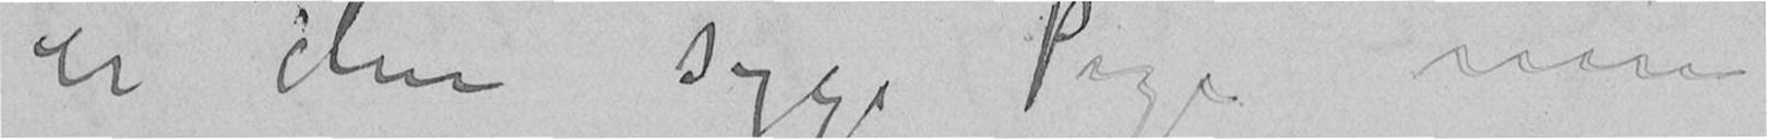er den syge Pige men
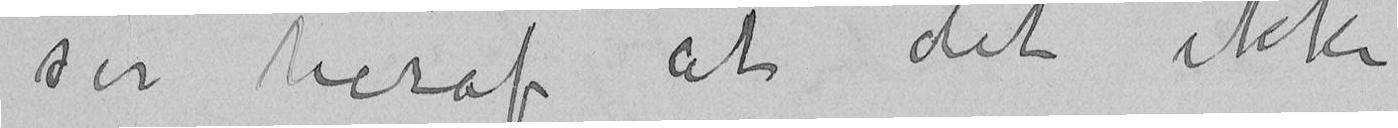ser heraf at det ikke
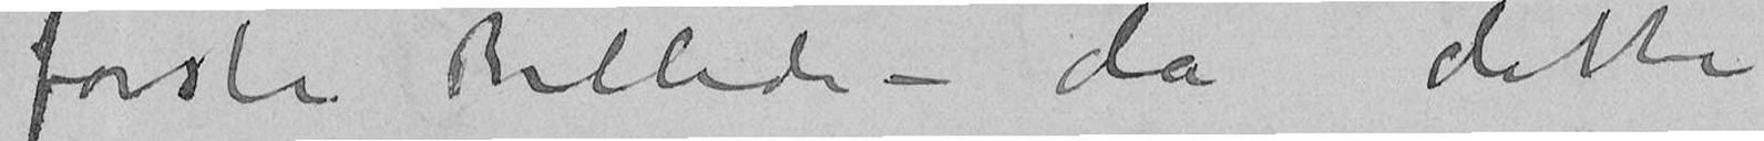første Billede – da dette
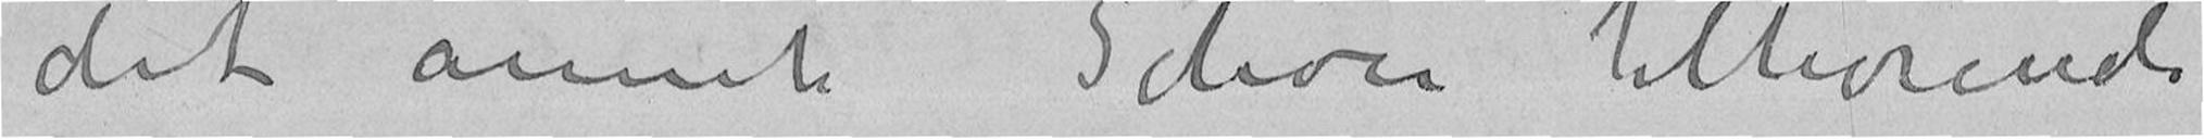det annet Schou tilhørende
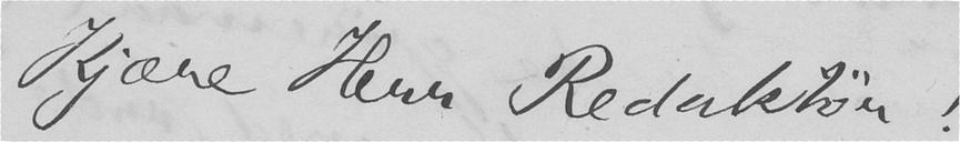Kjære Herr Redaktør!
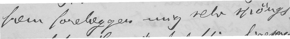frem forelægger mig selv spørgs-
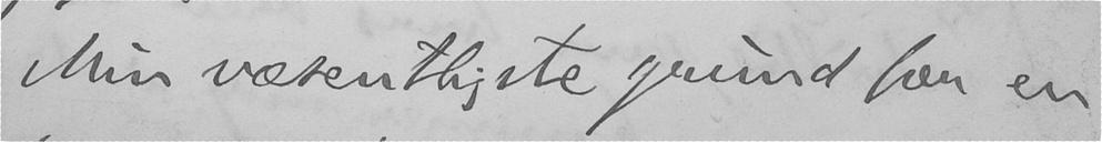Min væsentligste grund for en
